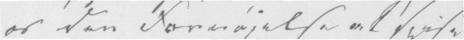os den Fornöjelse at spise
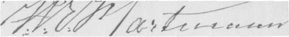J.P.E.Hartmann
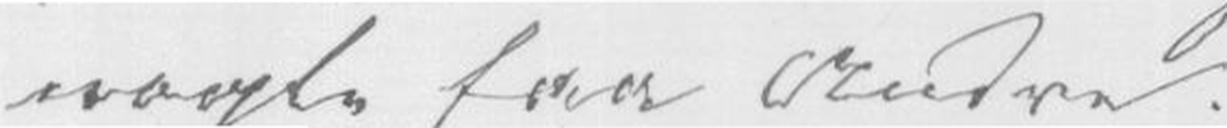nogle faa Andre.
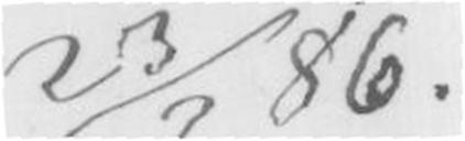23/2 86.
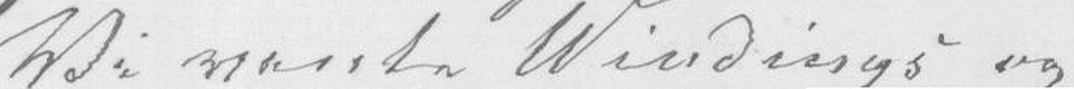Vi vente Windings og
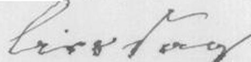Tirsdag
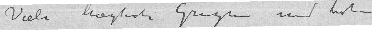Viele hirzlich Gruzsen und beste
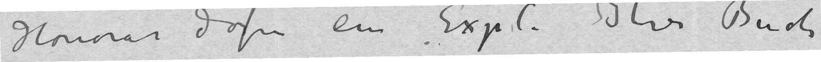Honorar dafur ein Expl. Ihr Buch
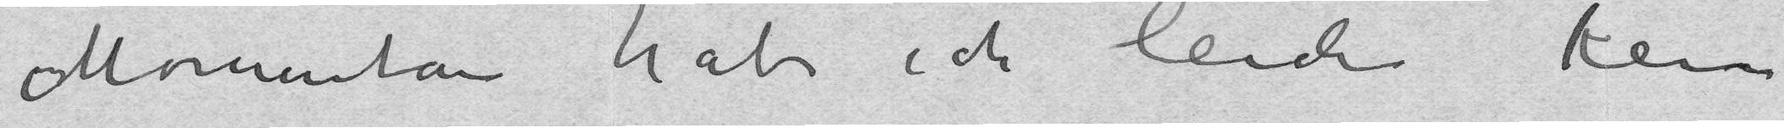Momentan habe ich leider kein
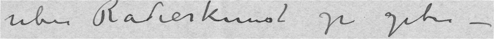uber Radierkunst zu geben –
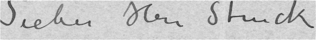Lieber Herr Struck
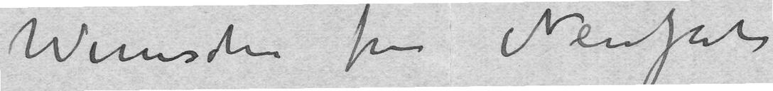Wunsche fur Neujahr
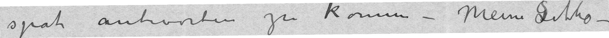spat antworten zu konnen – Meine Litho-
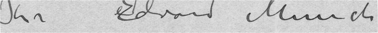Ihr Edvard Munch
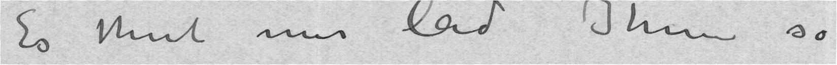Es thut mir leid Ihnen so
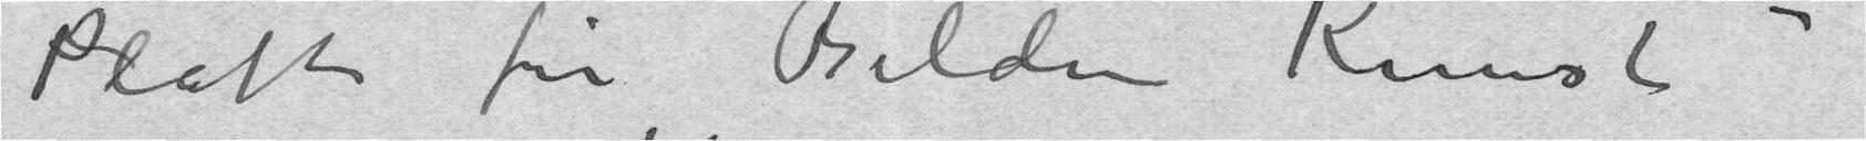Blatt für «Bilden Kunst»
